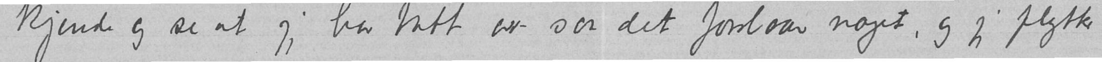kjende og se at jeg har tatt av saa det forslaar noget, og jeg flytter
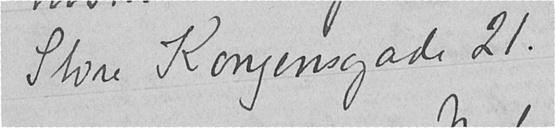Store Kongensgade 21.
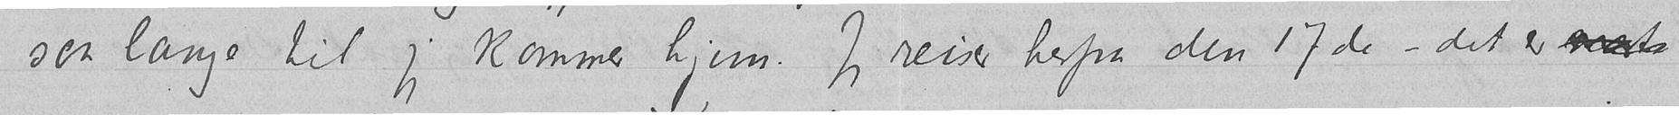saa længe til jeg kommer hjem. Jeg reiser herfra den 17de – det er
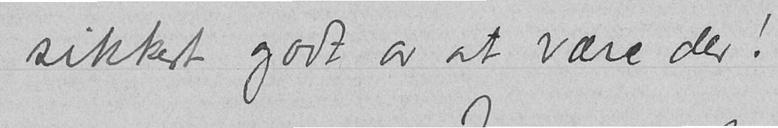sikkert godt av at være der!
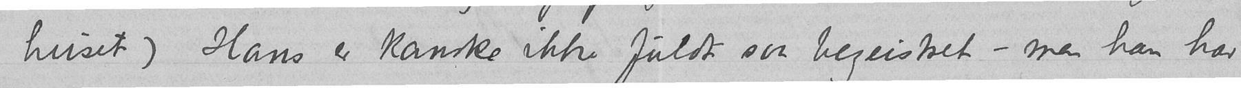huset) Hans er kanske ikke fuldt saa begeistret – men han har
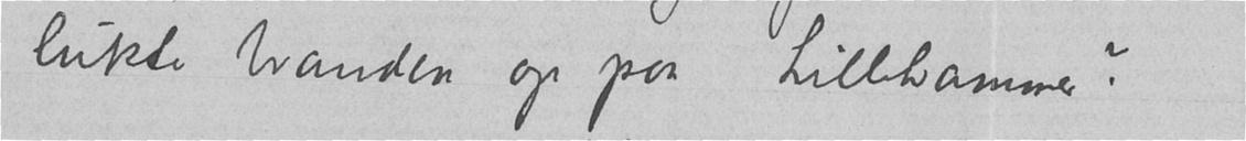lukte branden op paa Lillehammer?
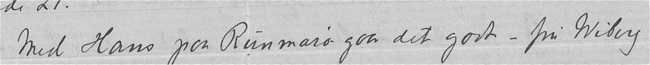Med Hans paa Runmarø gaar det godt – fru Wiberg
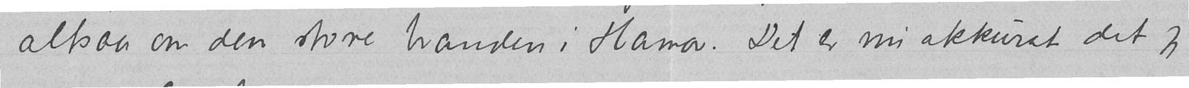altsaa om den store branden i Hamar. Det er nu akkurat det jeg
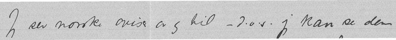Jeg ser norske aviser av og til – d.v.s. jeg kan se dem
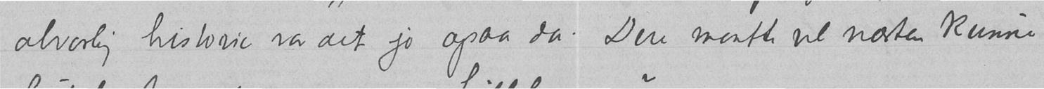alvorlig historie var det jo ogsaa da. Dere maatte vel næsten kunne
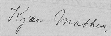Kjære Mathea,
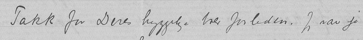Takk for Deres hyggelige brev forleden. Jeg var jo
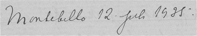Montebello 12. juli 1935.
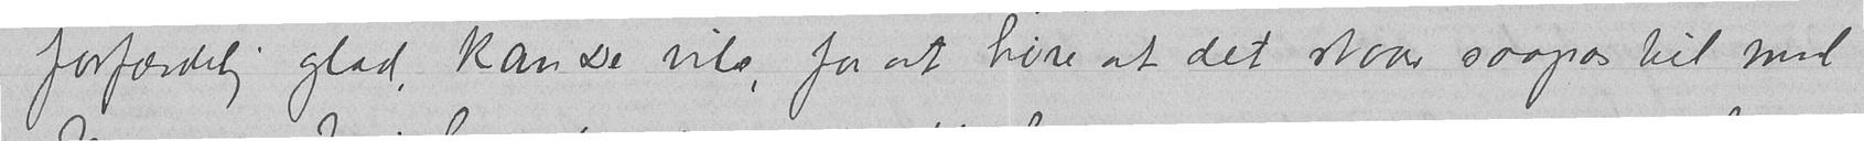forfærdelig glad, kan De vite, for at høre at det staar saapas til med
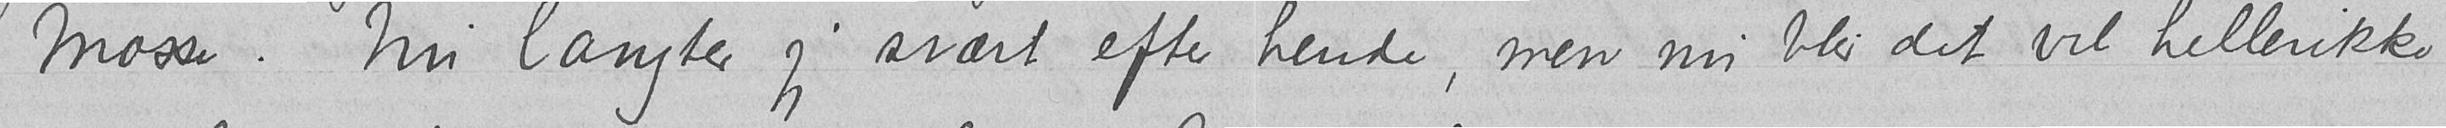Mosse. Nu længter jeg svært efter hende, men nu blir det vel hellerikke
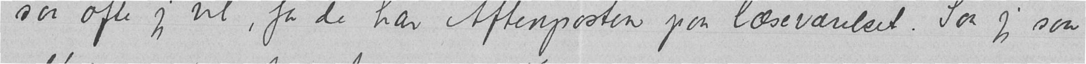saa ofte jeg vil, for de har Aftenposten paa læseværelset. Saa jeg saa
